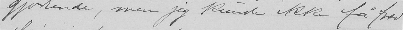gjørende, men jeg kunde ikke få fred
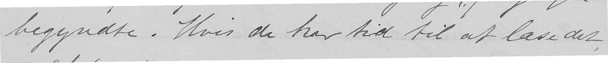begyndte. Hvis de har tid til at læse det,
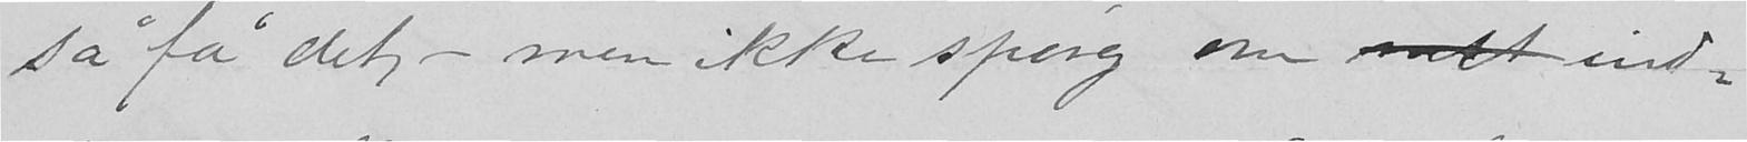så få det,- men ikke spørg om ind-
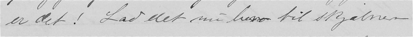er det! Lad det nu bero til skjebnen
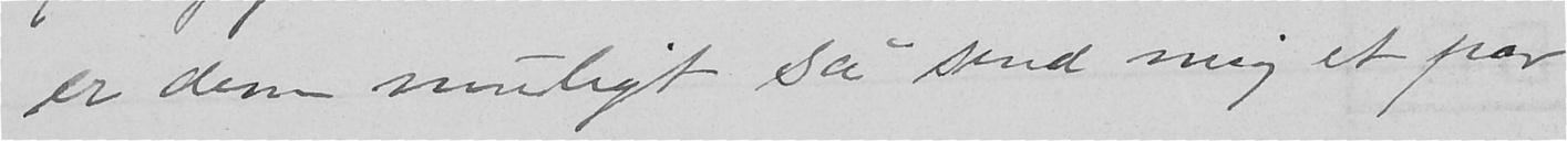er dem muligt så send mig et par
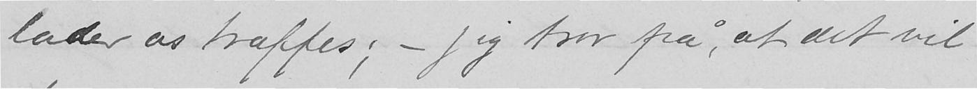lader os træffes; - jeg tror på, at det vil
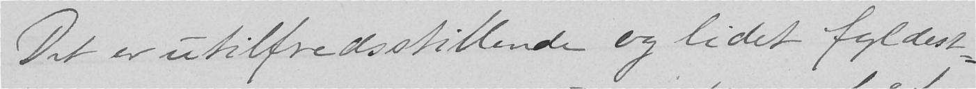Det er utilfredsstillende og lidet fyldest-
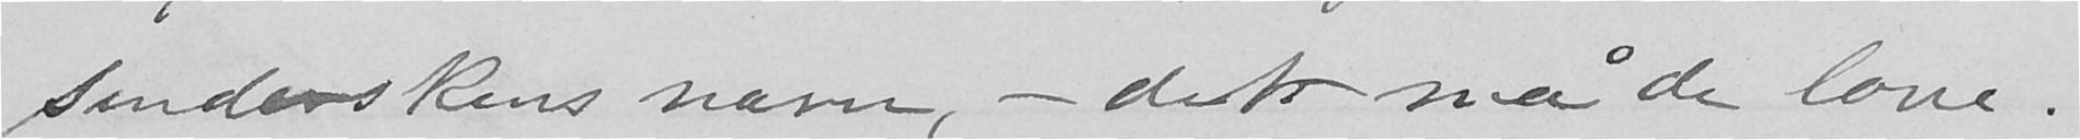senderskens navn, - det må de love.
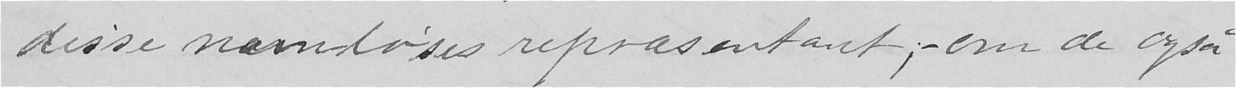disse navnløses representant,- om de også
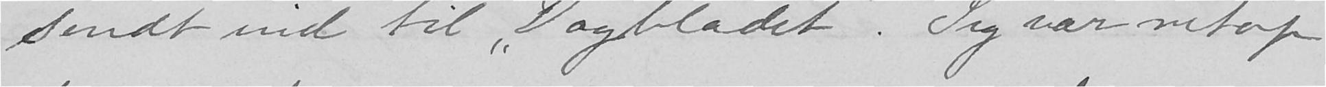sendt ind til "Dagbladet". Jeg var netop
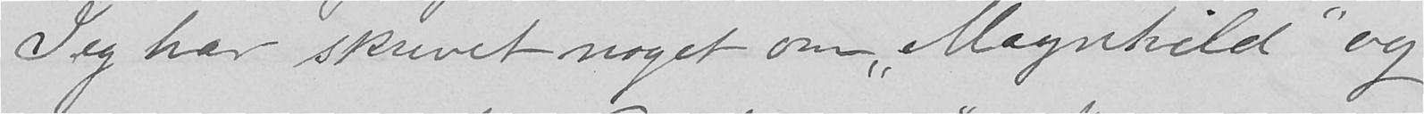Jeg har skrevet noget om "Magnhild" og
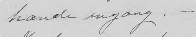hænde engang. -
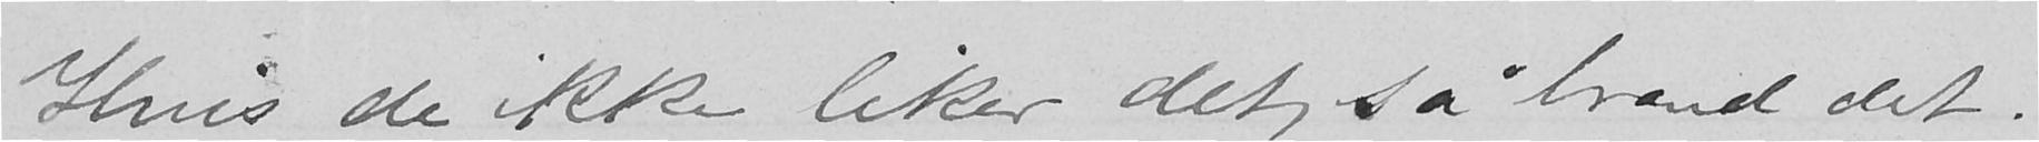Hvis de ikke liker det, så brænd det.
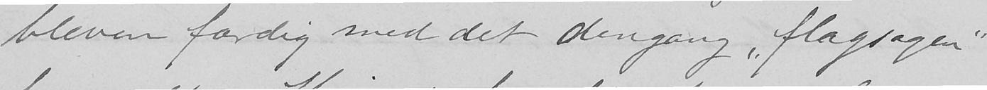bleven færdig med det dengang, "flagsagen"
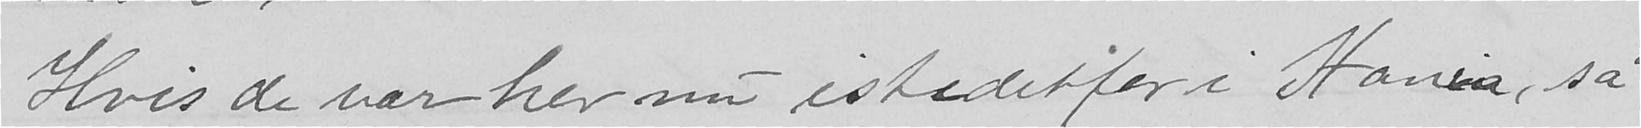Hvis de var her nu istedetfor i Xania, så
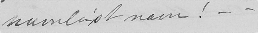navnløst navn! - -
